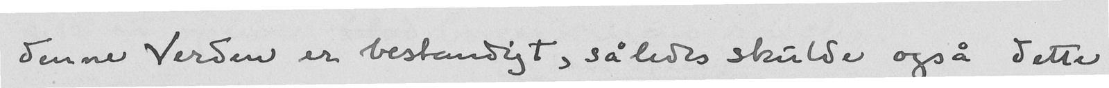denne Verden er bestandigt, således skulde også dette
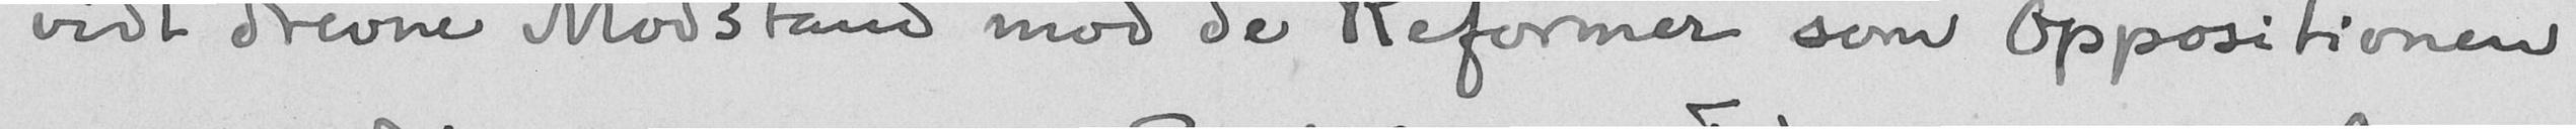vidt drevne Modstand mod de Reformer som Oppositionen
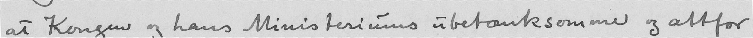at Kongen og hans Ministeriums ubetænksomme og altfor
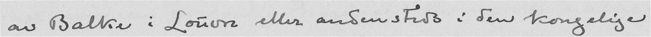av Balke i Louvre eller andensteds i den kongelige
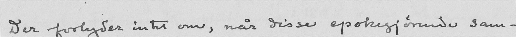Der forlyder intet om, når disse epokegjørende sam-
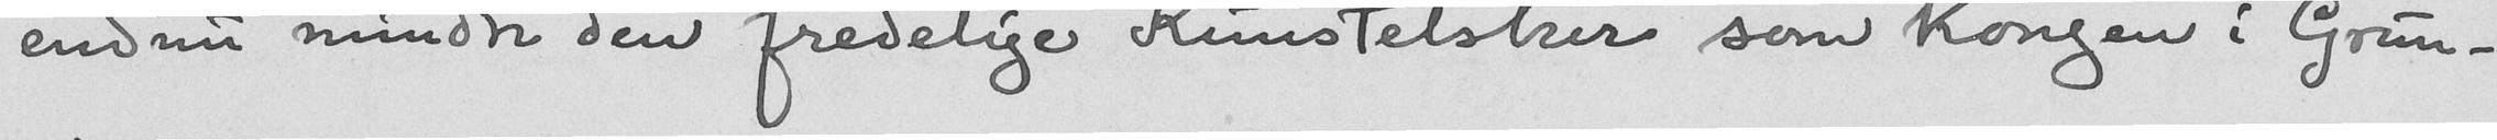endnu mindre den fredelige Kunstelsker som Kongen i Grun-
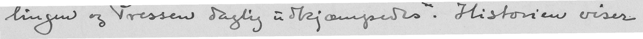lingen og Pressen daglig udkjæmpedes. Historien viser
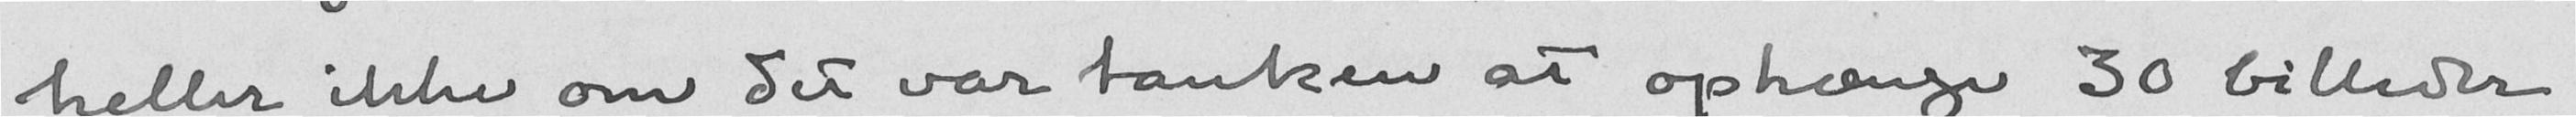heller ikke om det var tanken at ophænge 30 billeder
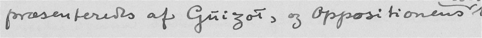præsenteredes af Guizot, og Oppositionens
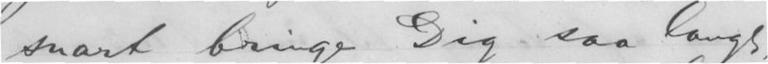snart bringe Dig saa langt,
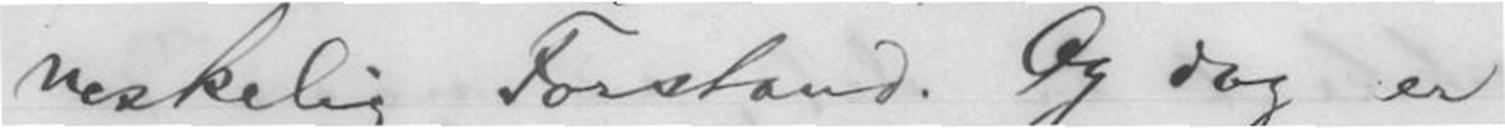neskelig Forstand. Og dog er
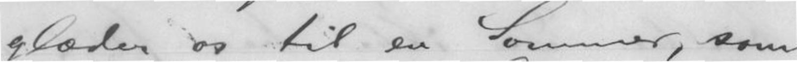glæder os til en Sommer, som
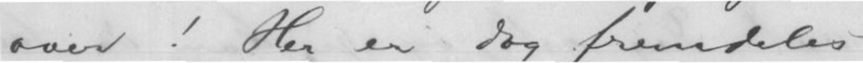over! Her er dog fremdeles
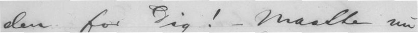den for Dig! - Maatte nu
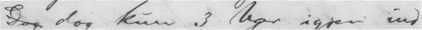Dog dog kun 3 Uger igjen ind-
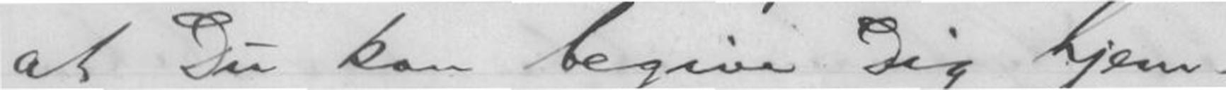at Du kan begive Dig hjem-
